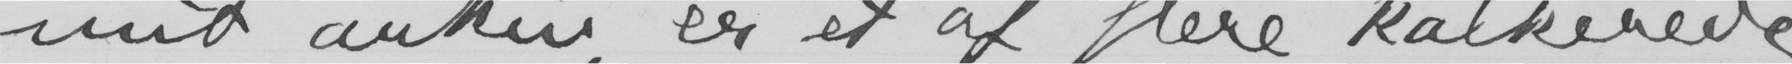mit arkiv, er et af flere kalkerede
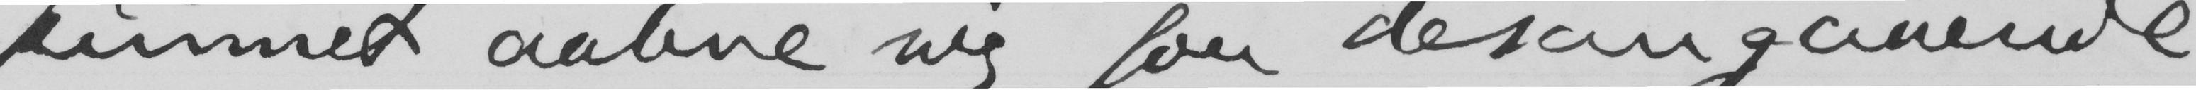kunnet aabne mig for desangaaende.
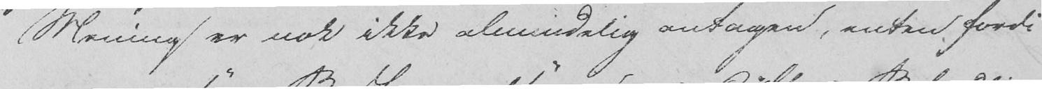Mening er nok ikke almindelig antagen, enten fordi
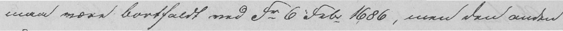maa være bortfaldt ved Fr 6 Febr 1686, men den anden
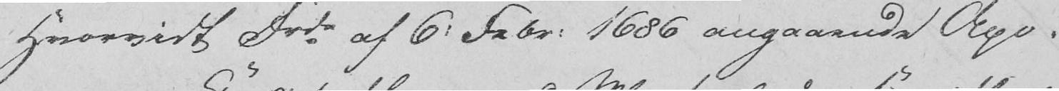Hvorvidt Frdn af 6. Febr. 1686 angaaende Apo-
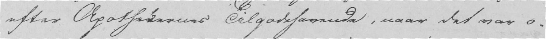efter Apothekernes Tilgodehavende, naar det var o-
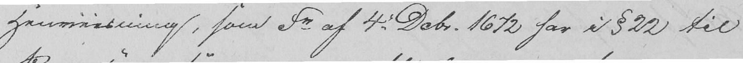Henviisning, som Fr af 4. Dcbr. 1672 har i § 22 til
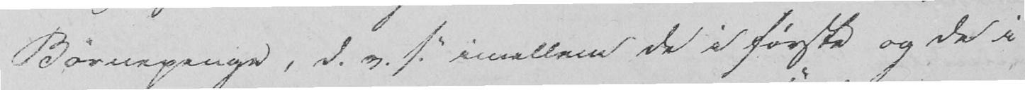Børnepenge, d. v. s. imellem de i første og de i
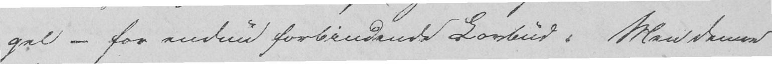gel - for endnu forbindende Lovbud. Men denne
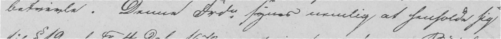betvivle. Denne Frdn synes nemlig at henholde sig
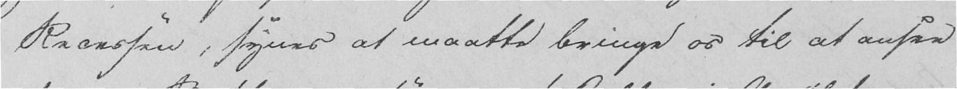Recessen, synes at maatte bringe os til at ansee
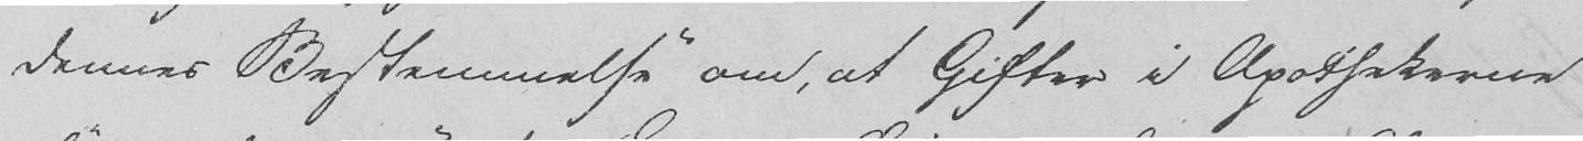dennes Bestemmelse om, at Gifter i Apothekerne
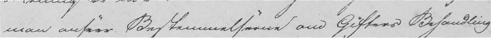man anseer Bestemmelserne om Gifters Behandling
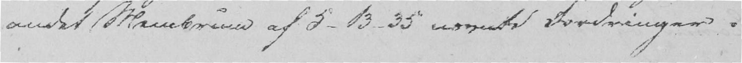andet Membrum af 5-13-35 nævnte Fordringer.
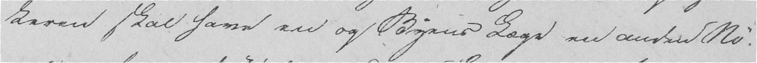keren skal have en og Byens Læge en anden Nø-
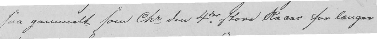saa gammelt som Chr den 4des store Reces for længer
